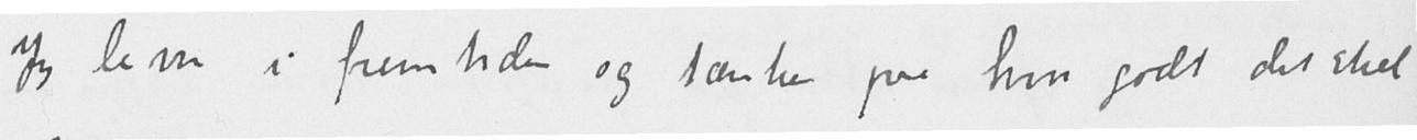Jeg lever i fremtiden og tænker paa hvor godt det skal
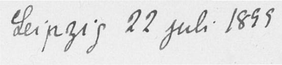Leipzig 22 juli 1899
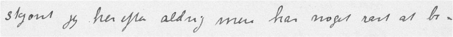skjønt jeg herefter aldrig mere har noget rart at be-
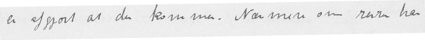er afgjort at du kommer. Nærmere om reisen har
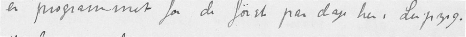er programmet for de første par dage her i Leipzig.
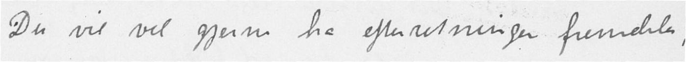Du vil vel gjerne ha efterretninger fremdeles,
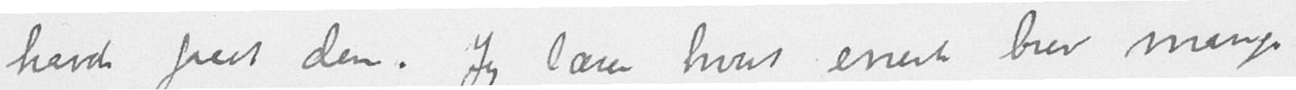havde faaet dem. Jeg læser hvert eneste brev mange
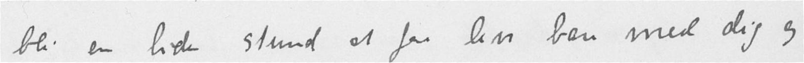bli en liden stund at faa leve bare med dig og
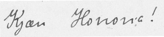Kjære Honoria!
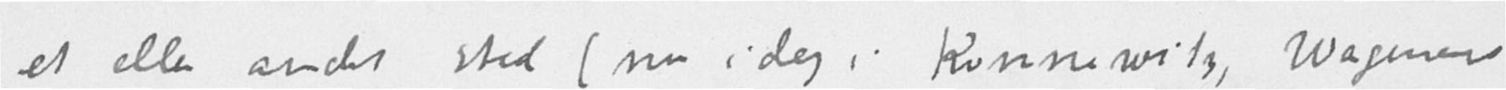et eller andet sted (nu i dag i Konnewitz, Wageners
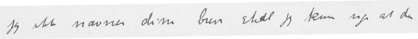jeg ikke nævner dine breve skal jeg kun sige at du
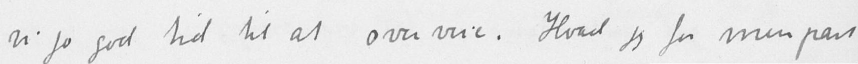vi jo god tid til at overveie. Hvad jeg for min part
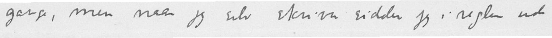gange, men naar jeg selv skriver sidder jeg i reglen ud
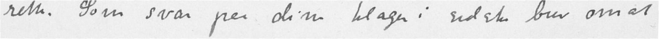rette. Som svar paa dine klager i sidste brev om at
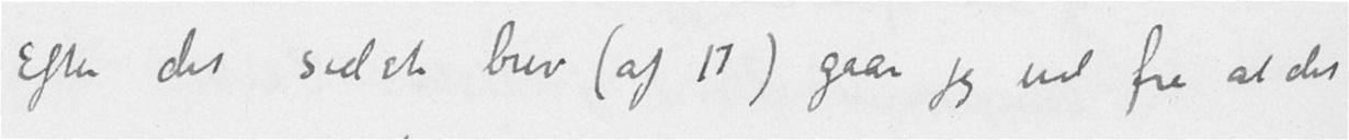Efter det sidste brev (af 17) gaar jeg ud fra at det
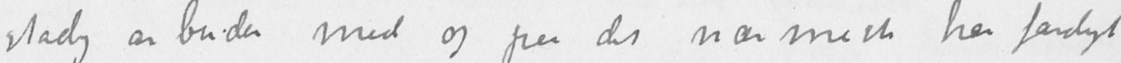stadig arbeider med og paa det nærmeste har færdigt
